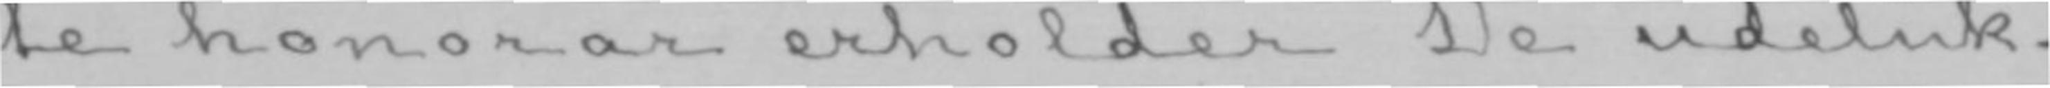te honorar erholder De udeluk-
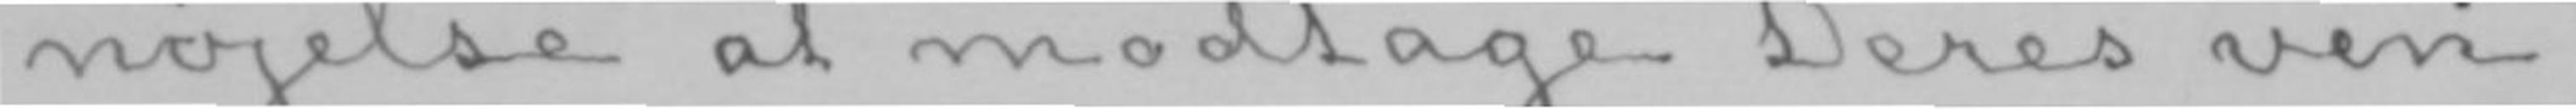nøjelse at modtage Deres ven-
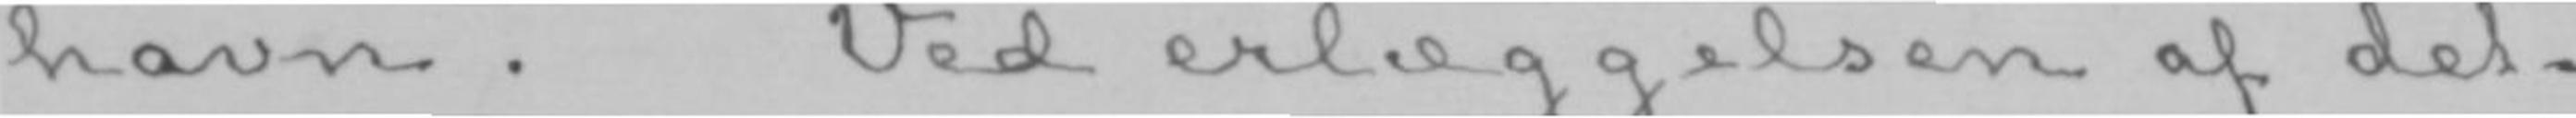havn. Ved erlæggelsen af det-
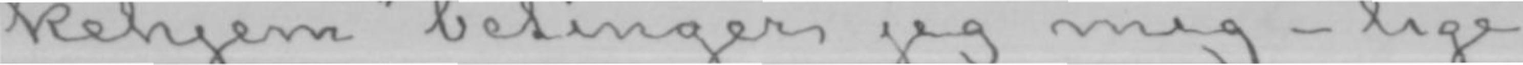kehjem» betinger jeg mig- lige-
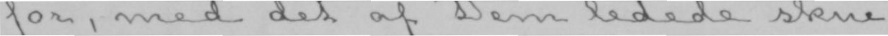for, med det af Dem ledede skue-
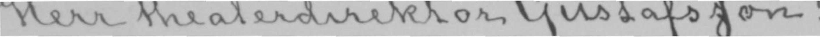Herr theaterdirektør Gustafsson!
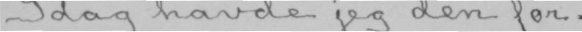Idag havde jeg den for-
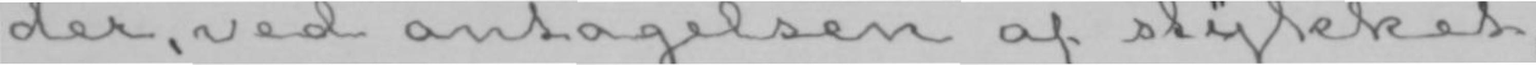der, ved antagelsen af stykket,
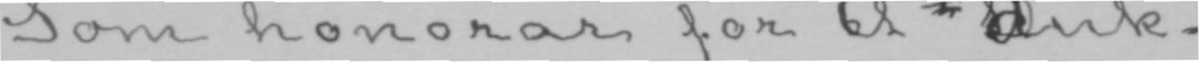Som honorar for «Et «duk-
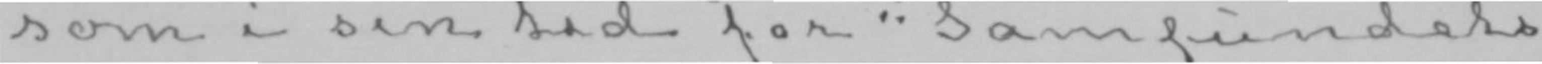som i sin tid for «Samfundets
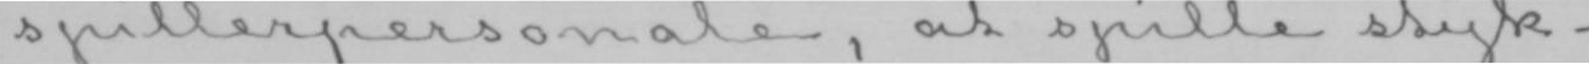spillerpersonale, at spille styk-
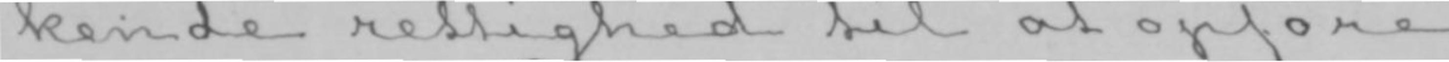kende rettighed til at opføre
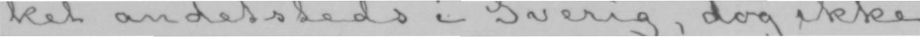ket andetsteds i Sverig, dog ikke
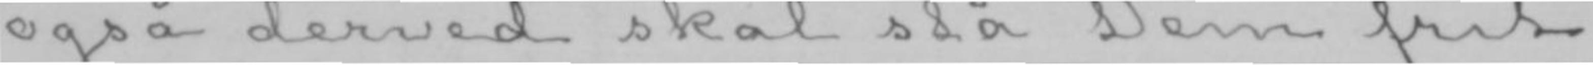også derved skal stå Dem frit
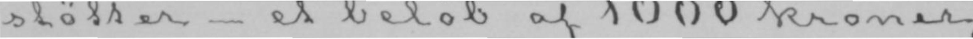støtter- et beløb af 1000 kroner,
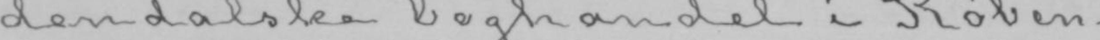dendalske boghandel i Køben-
